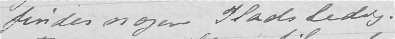findes nogen Plads ledig.
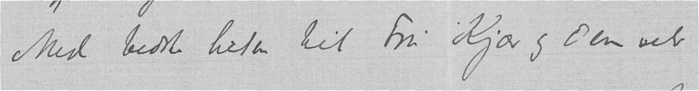Med bedste hilsen til Fru Kjær og Dem selv
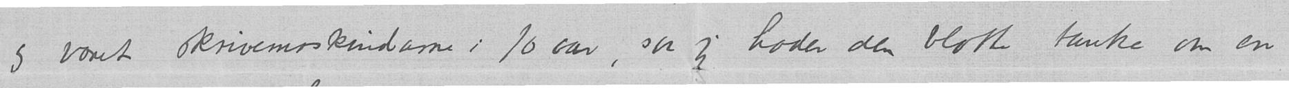og været skrivemaskindame i 10 aar, saa jeg hader den blotte tanke om en
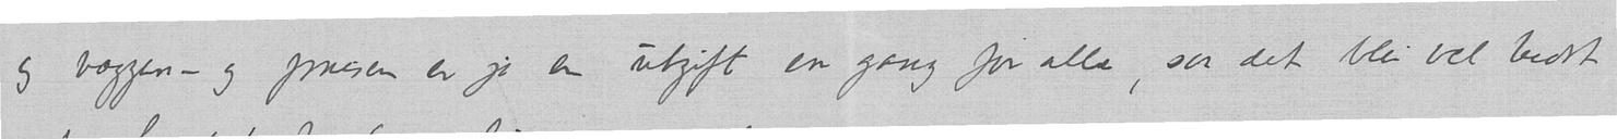og væggen – og prisen er jo en utgift en gang for alle, saa det blir vel bedst
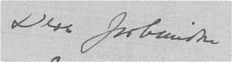Deres forbundne
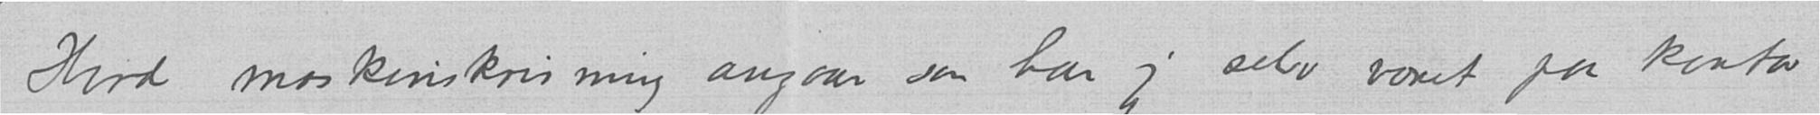Hvad maskinskrivning angaar saa har jeg selv været paa kontor
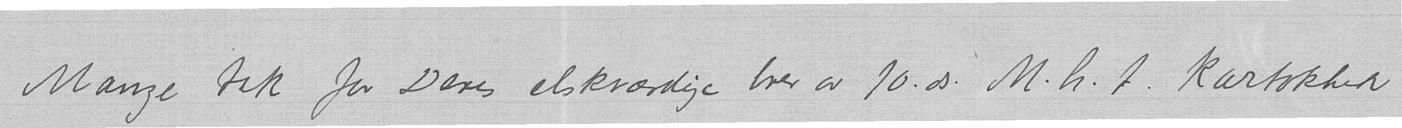Mange tak for Deres elskværdige brev av 10.ds. M.h.t. kartothek
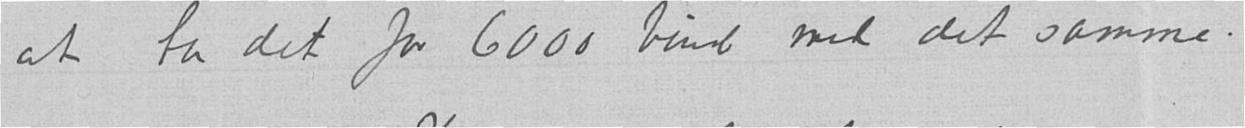at ta det for 6000 bind med det samme.
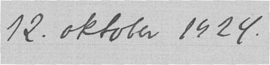12. oktober 1924.
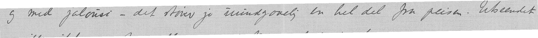og med jalousi – det støver jo uundgaaelig en hel del fra peisen. Utseendet
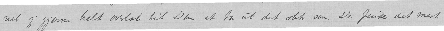vil jeg gjerne helt overlate til Dem at ta ut det slik som De finder det mest
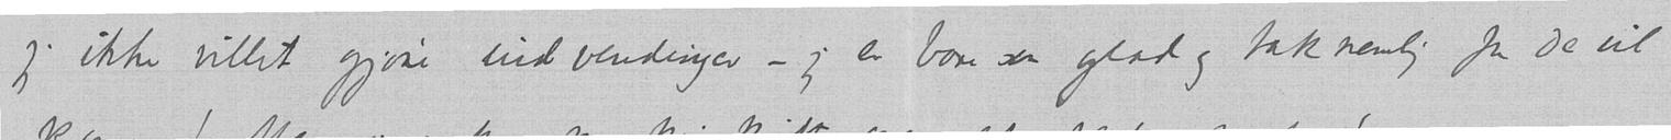jeg ikke villet gjøre indvendinger – jeg er bare saa glad og taknemlig for de vil
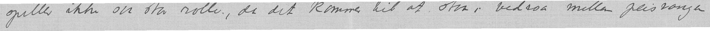spiller ikke saa stor rolle., da det kommer til at staa i vedroa mellem peisvangen
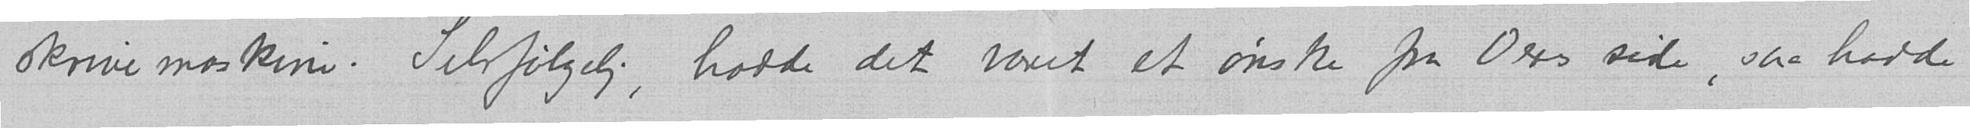skrivemaskine. Selvfølgelig, hadde det været et ønske fra Deres side, saa hadde
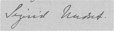Sigrid Undset.
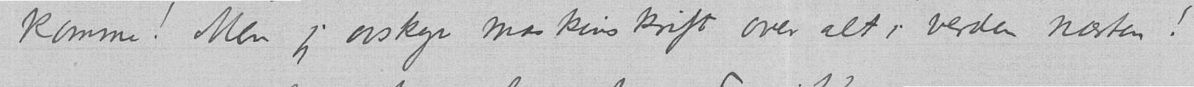komme! Men jeg avskyr maskinskrift over alt i verden næsten!
